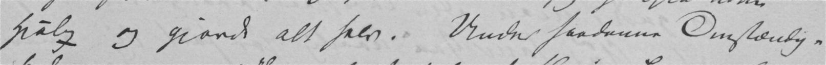Hjelp og gjorde alt selv. Under saadanne Omstændigheder
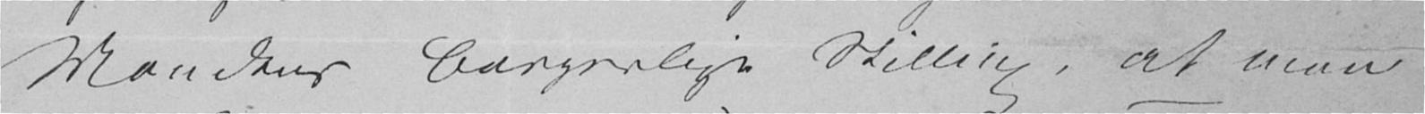Mandens borgerlige Stilling, at man
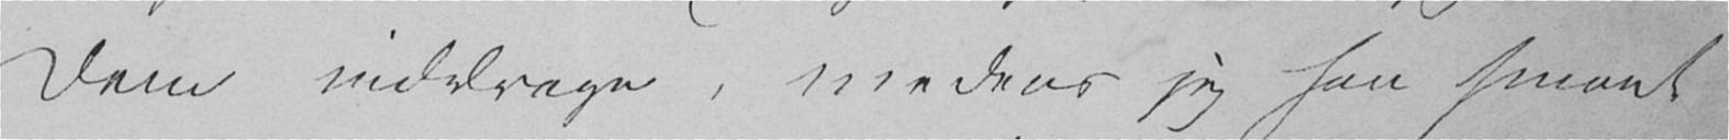Dem inddrage, medens jeg saa smaat
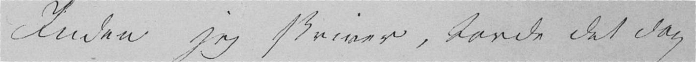Inden jeg skriver, torde det dog
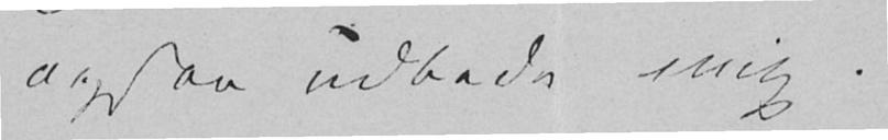ogsaa udbede mig.
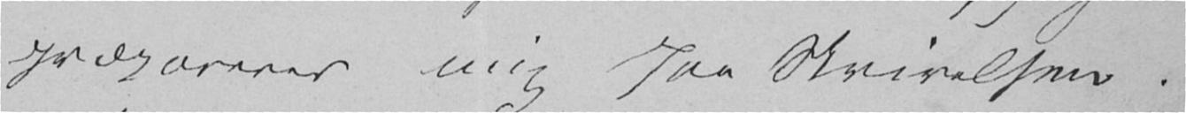præparerer mig paa Skrivelsen.
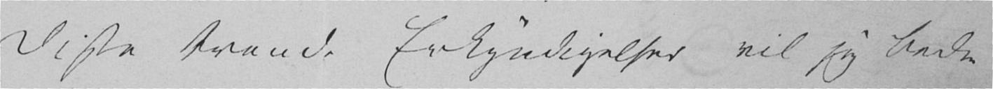Disse tvende Erkyndigelser vil jeg bede
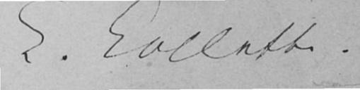C. Collett.
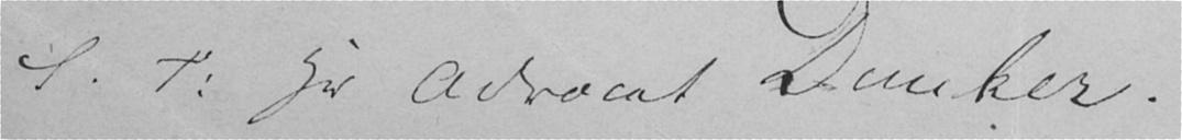S: T: Hr Advokat Dunker.
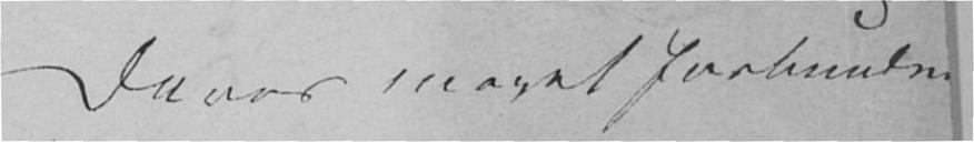Deres meget forbundne
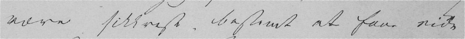være sikkrest, bestemt at faae vide
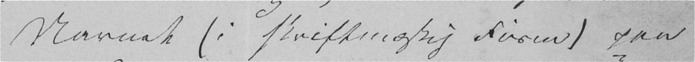Navnet (i Skriftmæssig Form) paa
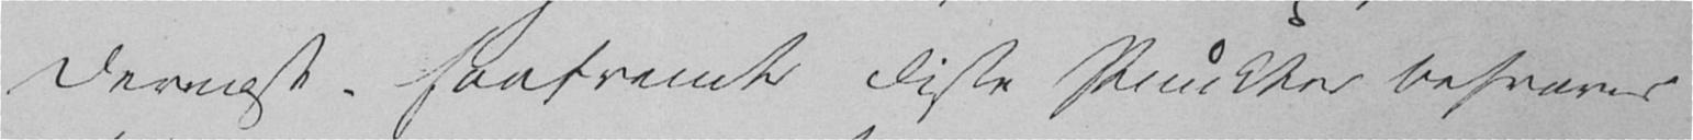Dernæst, saafremt disse Punkter besvares
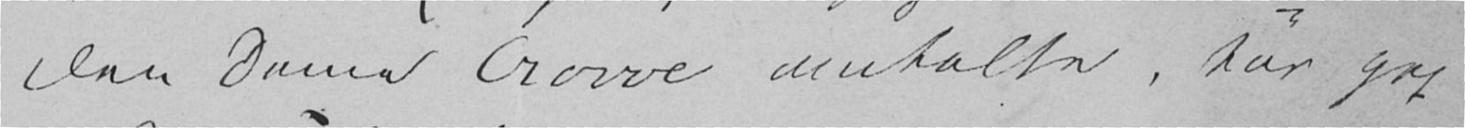den Dame Crowe omtalte, tør jeg
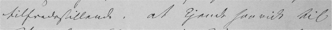tilfredstillende, at kjende saavidt til
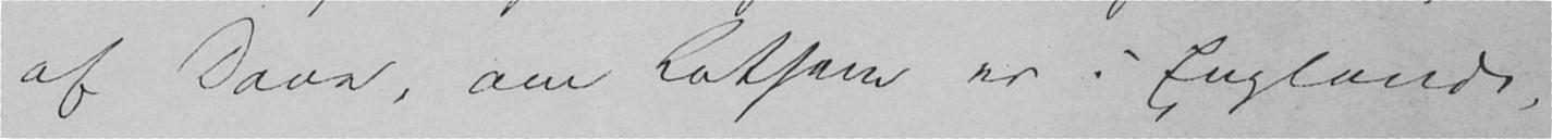af Daae, om Latham er i England,
